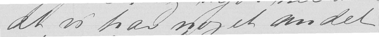at vi har noget andet
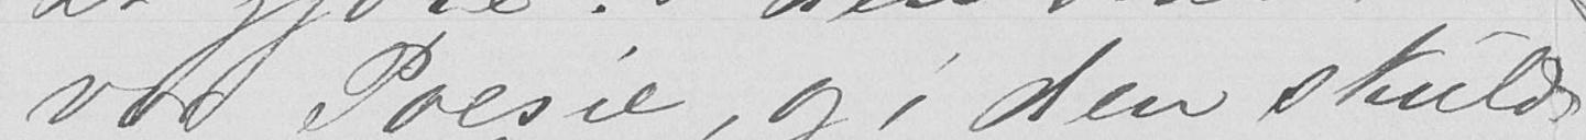vor Poesie, og i den skulde
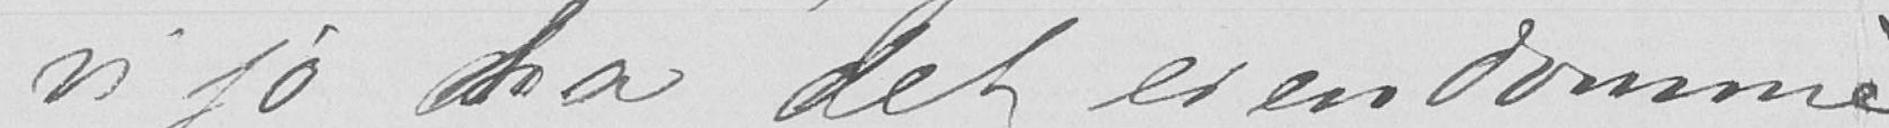vi jo ha det eiendomme-
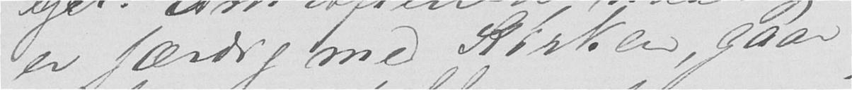er færdig med Kirken, gaar
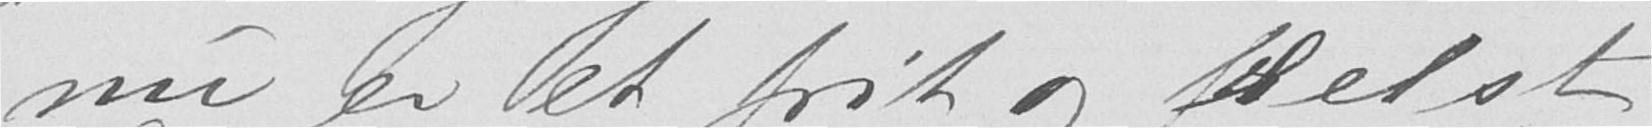nu er et frit og frelst
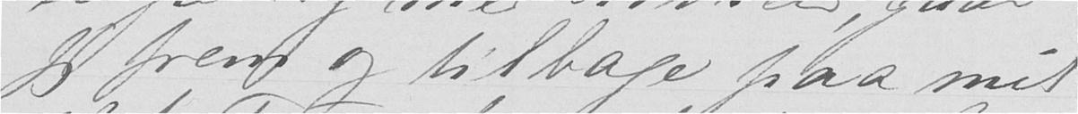jeg frem og tilbage paa mit
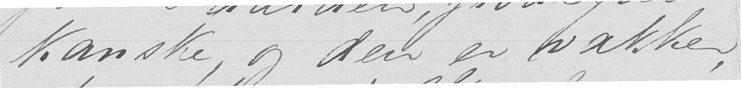kanske, og den er vakker
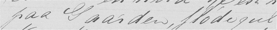paa Gaarden, flødegul
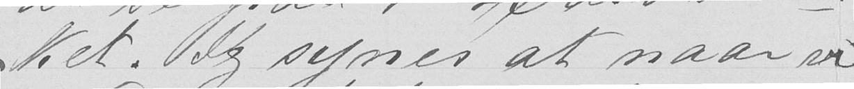ket. Jeg synes at naar vi
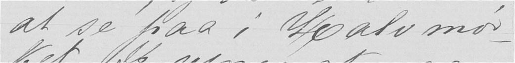at se paa i Halv mør-
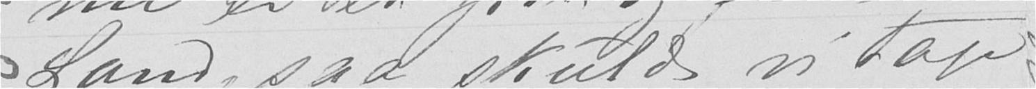Land, saa skulde vi tage
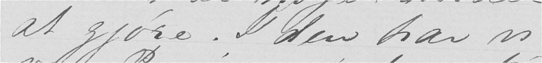at gjøre. I den har vi
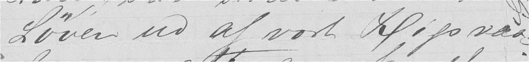Løven ud af vort Rigsvaa-
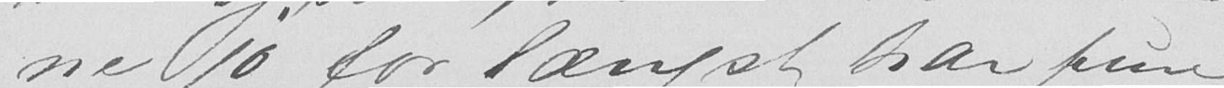ne jo for længst har fun-
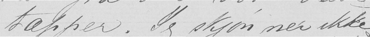tæpper. Jeg skjønner ikke
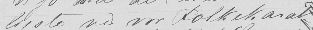ligste ved vor Folkekarak-
